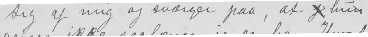sig af mig og sværger paa, at j hun
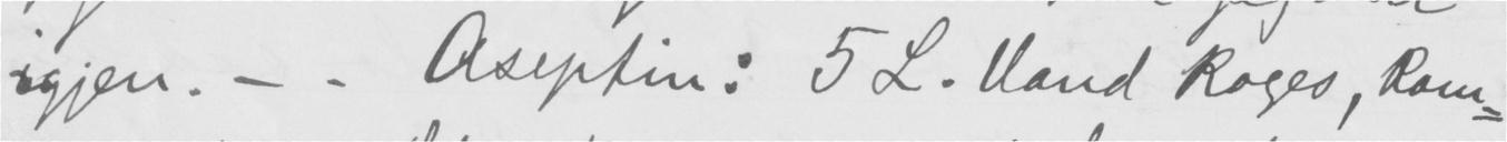igjen. — Aseptin: 5 L. Vand Roges, Rom-
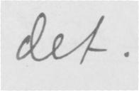det.
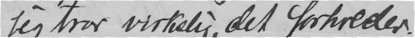jeg tror virkelig, det forholder
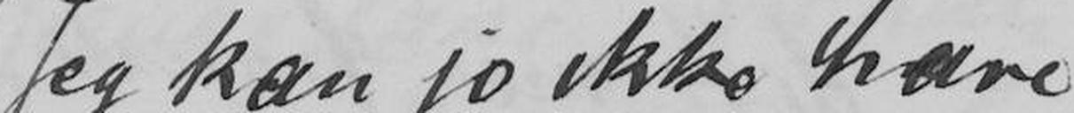Jeg kan jo ikke have
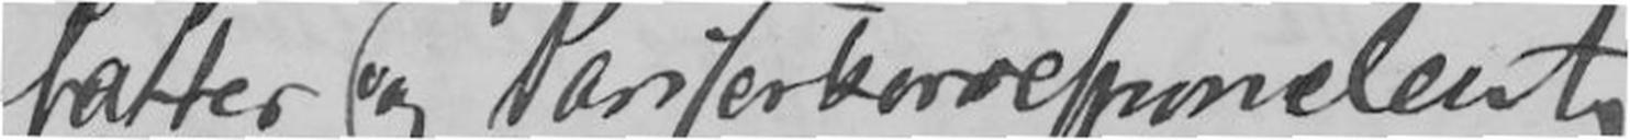fatter og Pariserkorrespondent
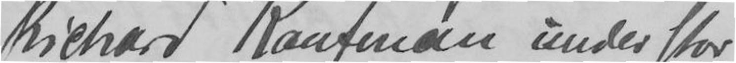Richard Kauferman under stor
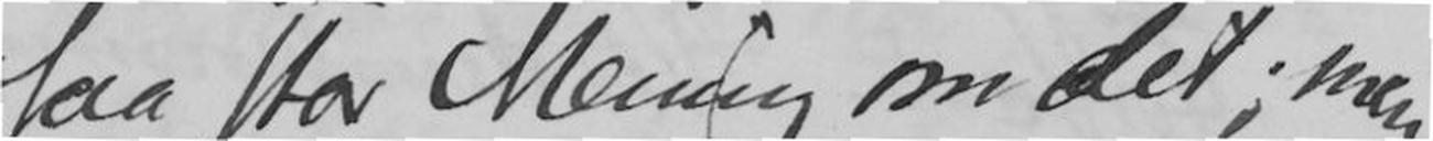saa stor Mening om det; men
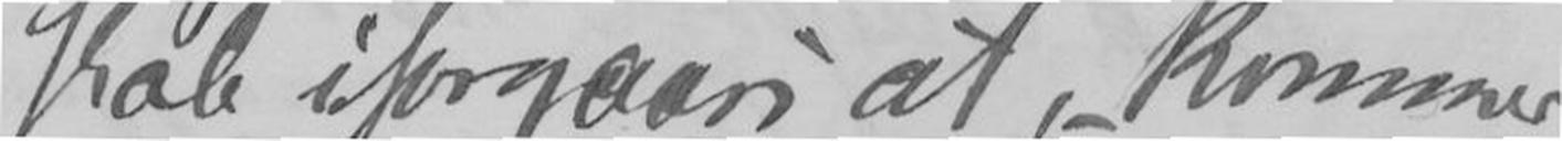skab iforgaars at, - kommer
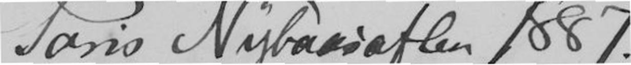Paris Nytaarsaften 1887.
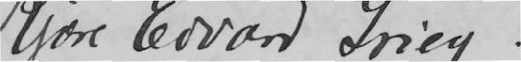Kjære Edvard Grieg!
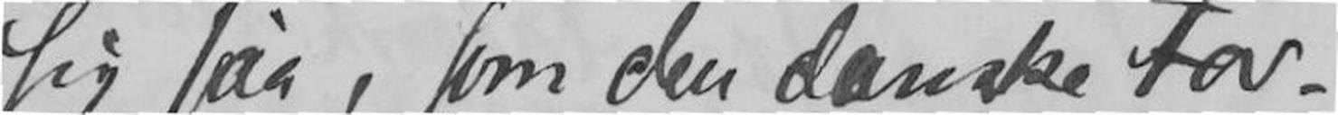sig saa, som den danske for-
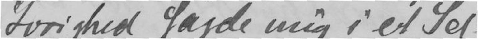Ivrighed Sagde mig i et Sel-
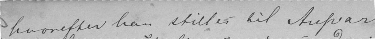hvorefter han stilles til Ansvar
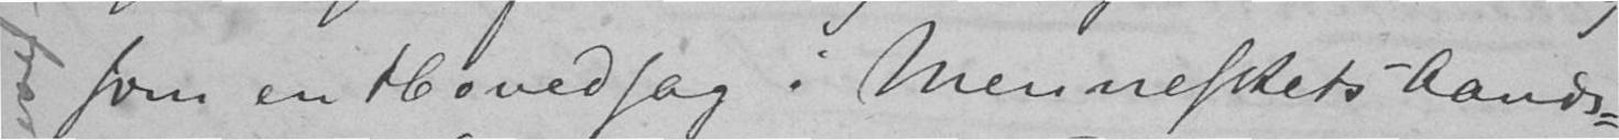som en Hovedsag. Menneskets Aands-
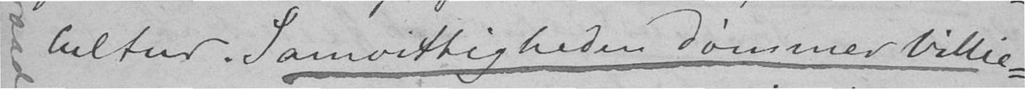cultur. Samvittigheden dømmer Villie-
